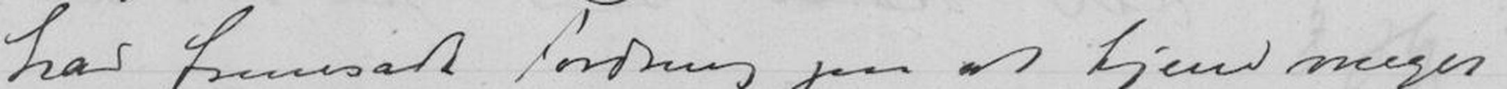har fremsadt Fordring paa at tjene meget
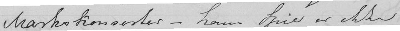Markskonserter - hans Spil er ikke
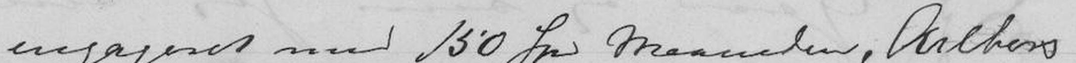engageret med 150 spd Maaneden. Arlberg
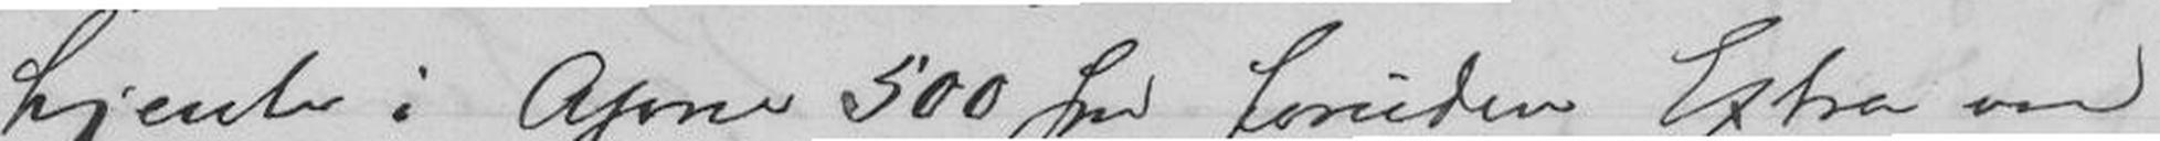tjente i April 500 spd foruden Extra ved
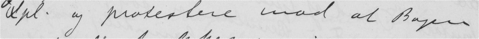Expl. og protestere mod at Bogen
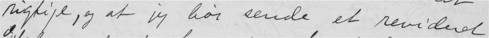rigtige, og at jeg bør sende et revideret
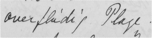overflødig Plage.
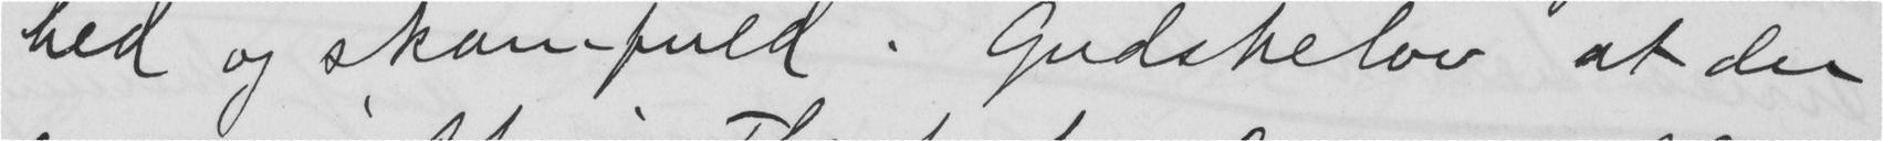hed og skamfuld. Gudskelov at der
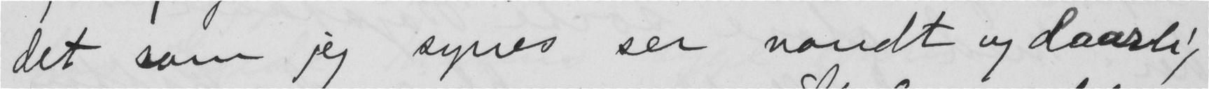det som jeg synes ser vondt og daarlig
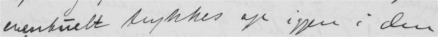eventuelt trykkes op igjen i den
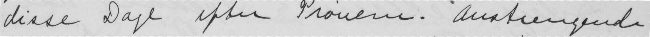disse Dage efter Prøvene. Anstrengende
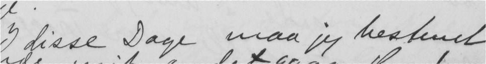I disse Dage maa jeg bestemt
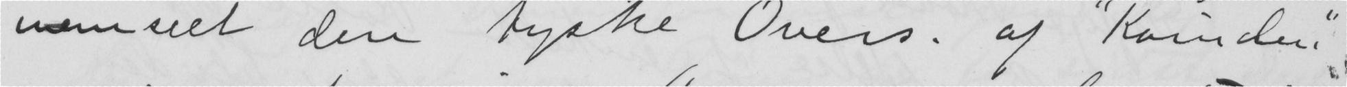nemseet den tyske Overs. af "Kvinden"
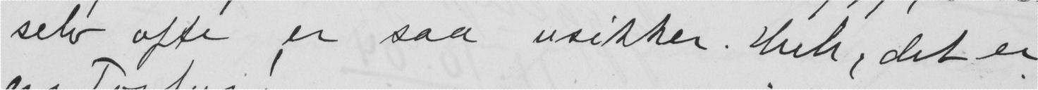selv ofte er saa usikker. Huh, det er
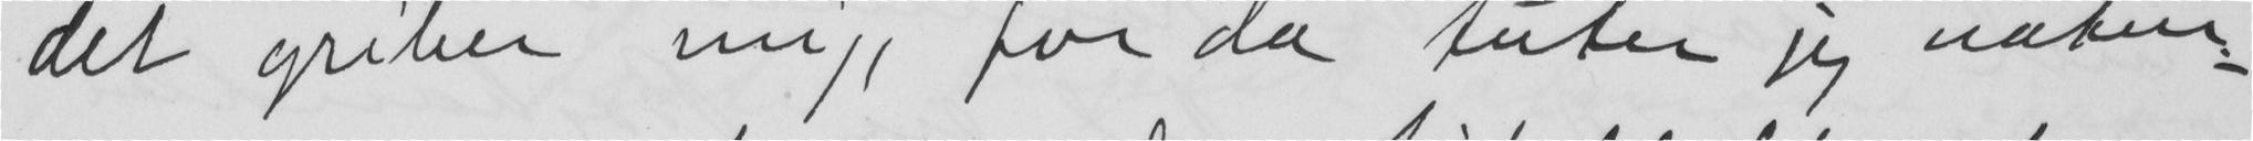det griber mig, for da tuter jeg natur-
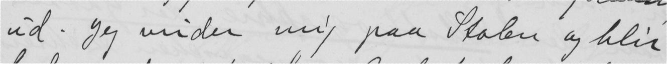ud. Jeg vrider mig paa Stolen og blir
